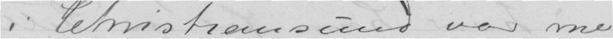i Christiansund var me
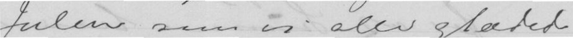Julen som vi alle glædede
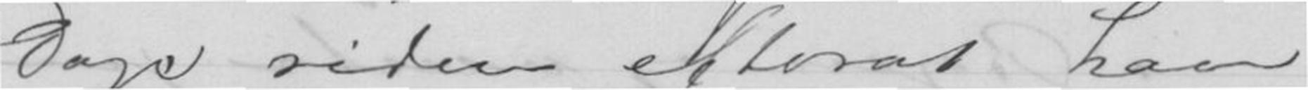Dage siden efterat have
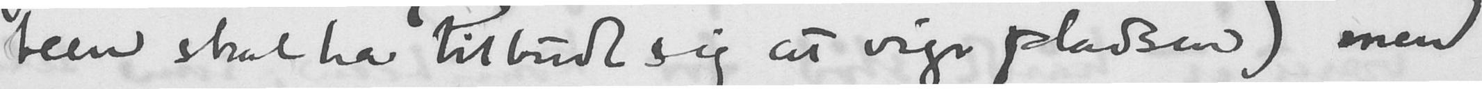teen skal ha tilbudt sig at vige pladsen) men
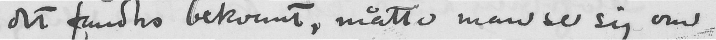det fandtes bekvemt, måtte man se sig om
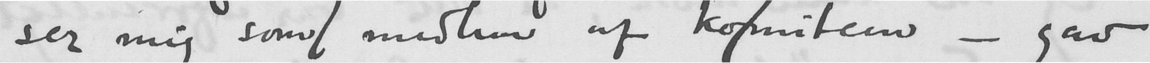ser mig som medlem af komiteen - gav
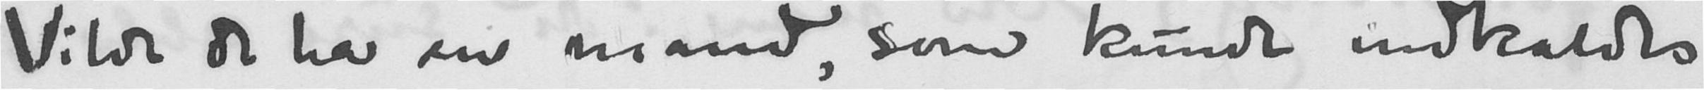Vilde de ha en mand, som kunde indkaldes
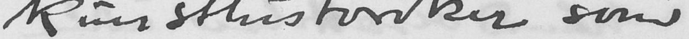kunsthistoriker som
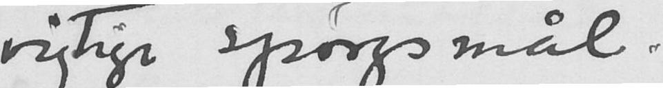vigtige spørgsmål.
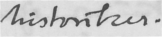historiker.
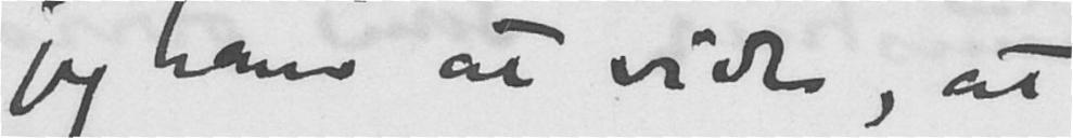jeg ham at vide, at
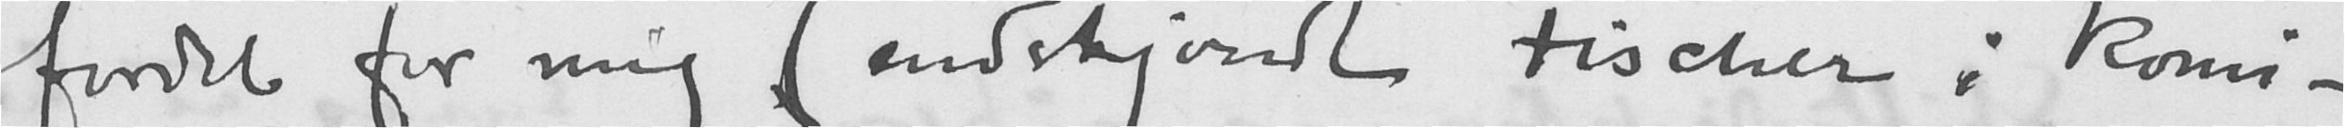fordel for mig, (endskjøndt Fischer i komi-
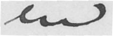en
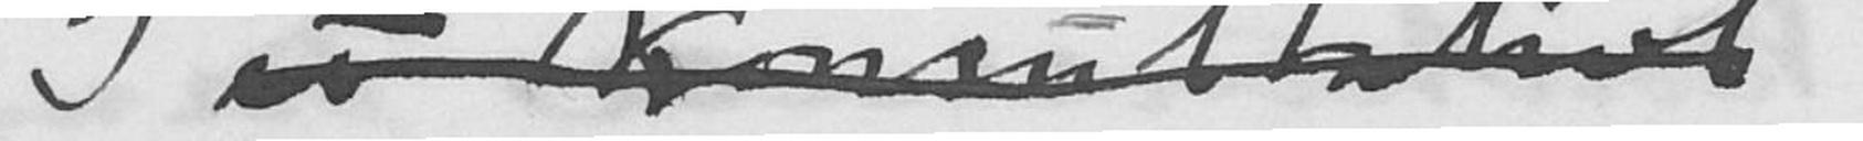1) et konsultativt
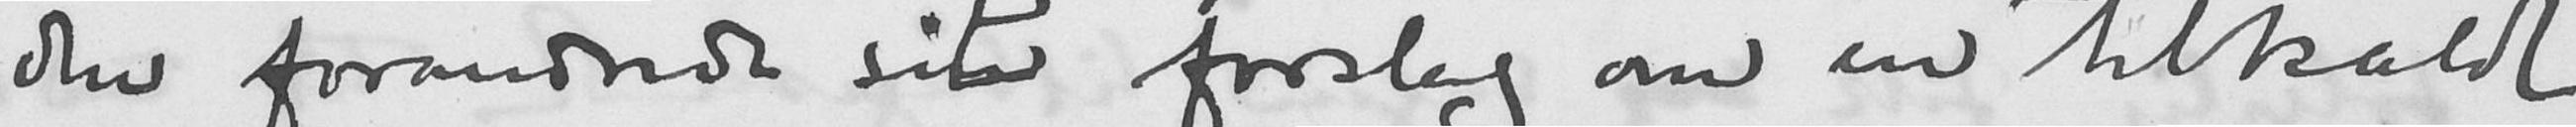den forandredt sit forslag om en tilkaldt
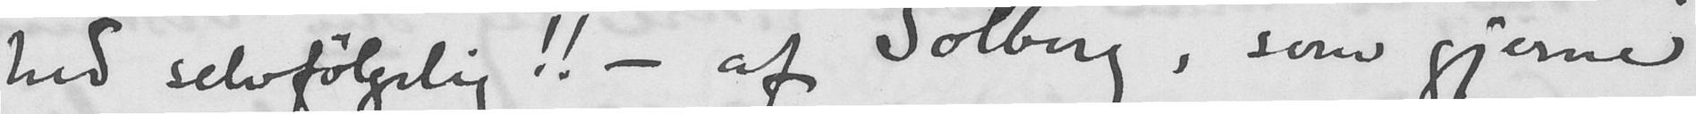hed selvfølgelig!! - af Solberg, som gjerne
